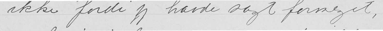- ikke fordi jeg havde sagt formeget,
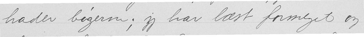hader bøgerne; jeg har læst for meget og
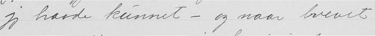jeg havde kunnet - og naar brevet
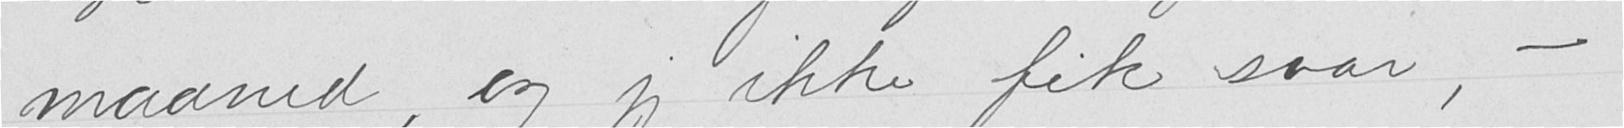maaned, og jeg ikke fik svar, -
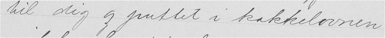til dig og puttet i kakkelovnen
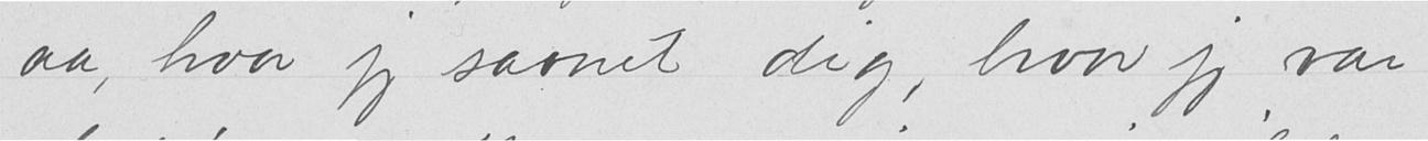aa, hvor jeg savnet dig, hvor jeg var
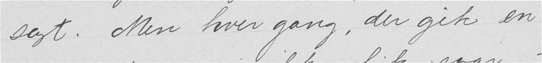sagt. Men hver gang, der gik en
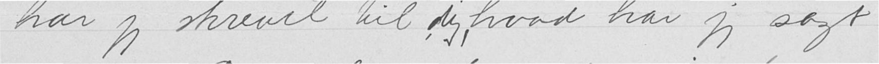har jeg skrevet til dig, hvad har jeg sagt
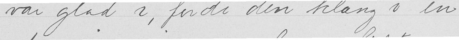var glad i, fordi den klang i en
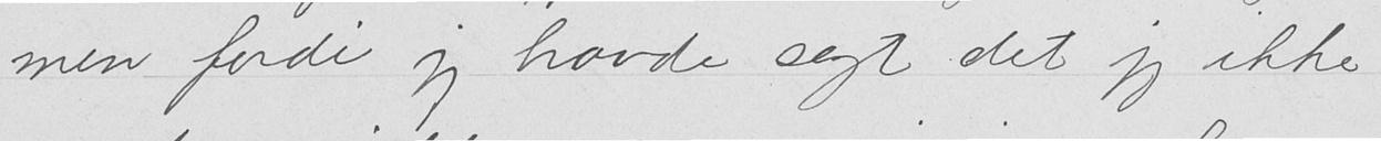men fordi jeg havde sagt det jeg ikke
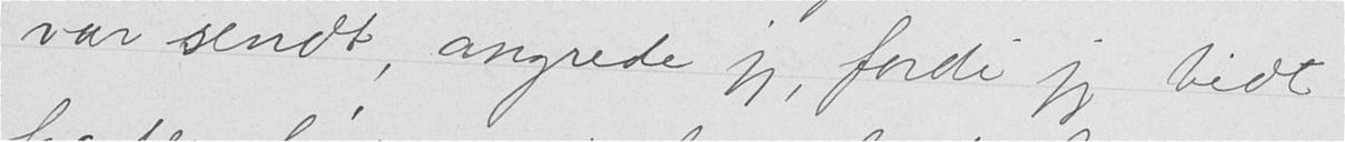var sendt, angrede jeg, fordi jeg bidt
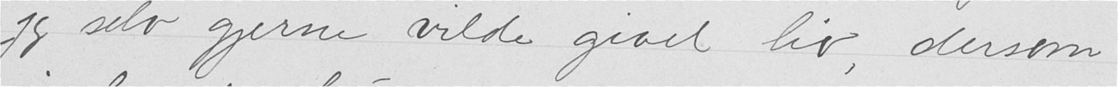jeg selv gjerne vilde givet liv, dersom
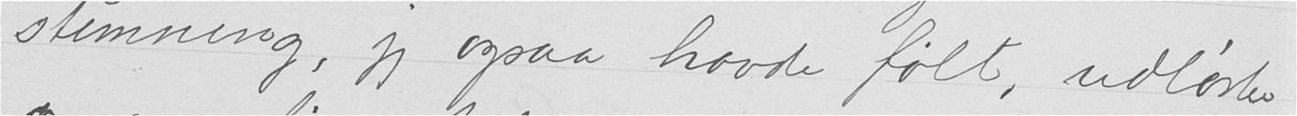stemning, jeg ogsaa havde følt, udløste
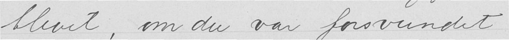blevet, om du var forsvundet -
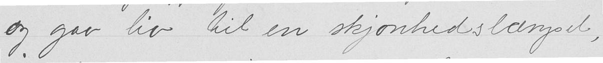og gav liv til en skjønhetslængsel,
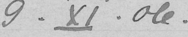9.XI.06.
